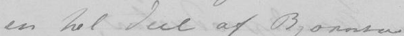en hel deel af Bjørnson
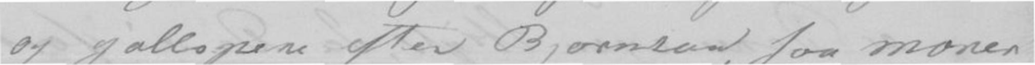og gallspere efter Bjørnson, saa morer
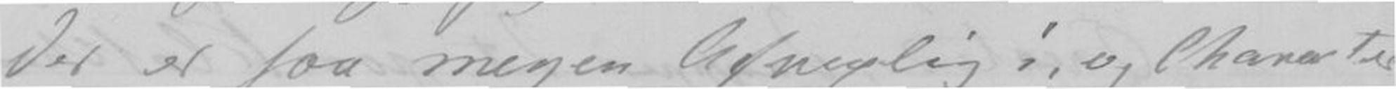der er saa megen Afneglig i, og Charater
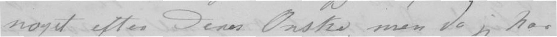noget efter Deres Ønske, men Da jeg har
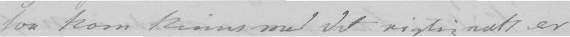saa kom kinns med det rigtig nok er
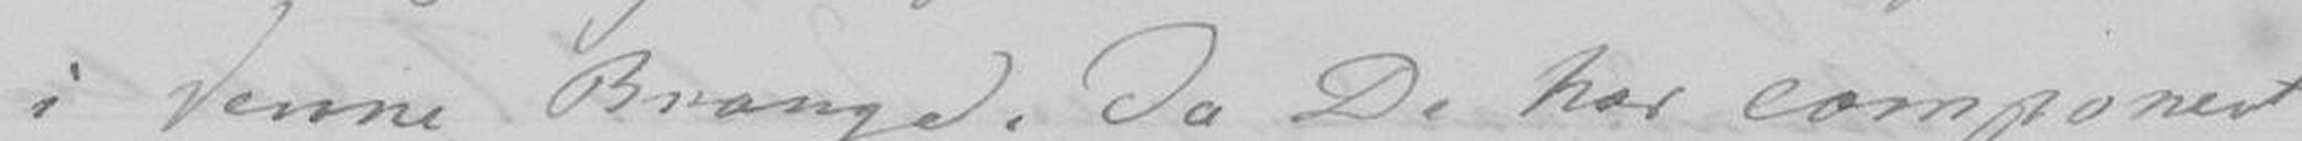i denne Brange. Da De har componert
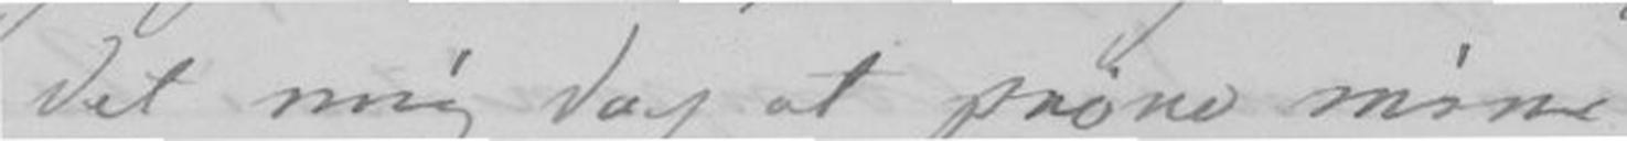det mig dog at prøve mine
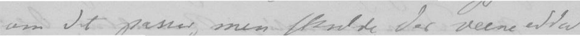om det passer, men skulde der veere er da
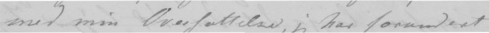med min Oversættelse, jeg har forandret
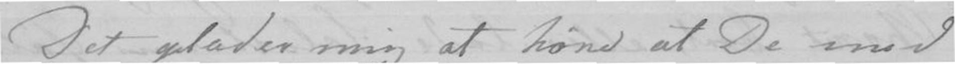Det glæder mig at høre at De med
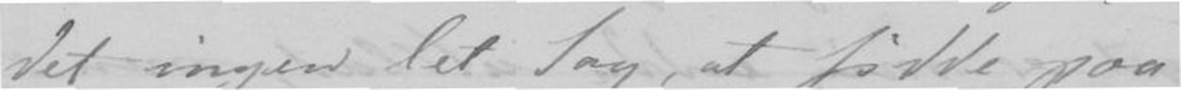det ingen let Sag, at sidde paa
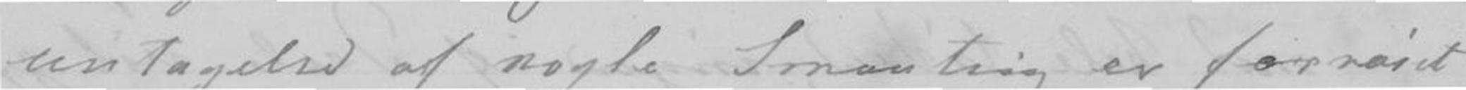untagelse af nogle Smaating er fornøiet
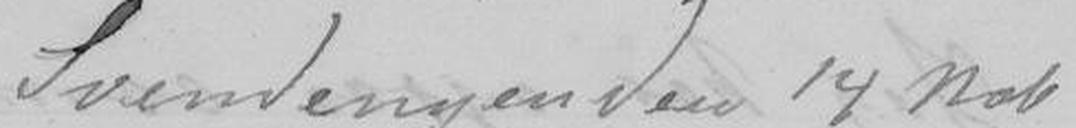Svendengenden 14 Nob
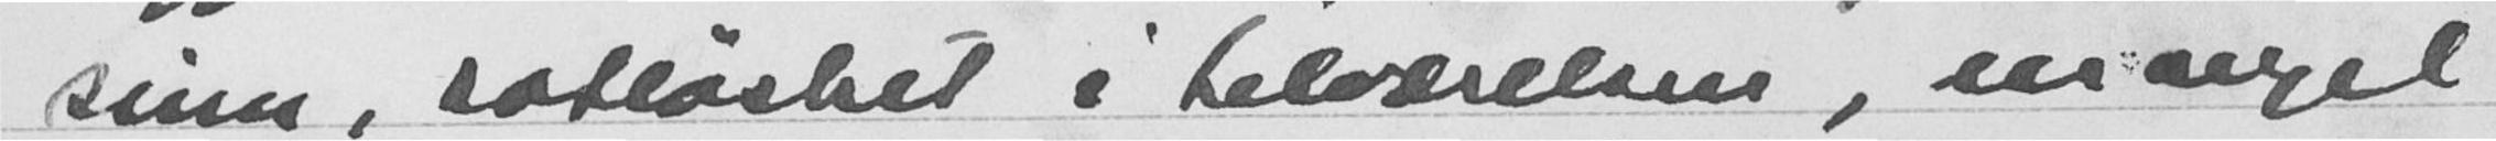sinn, rotløshet i tilværelsen, mangel
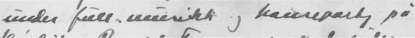under full musikk og houseparty på
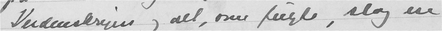Verdenskrigen og alt, som fulgte, slog en
